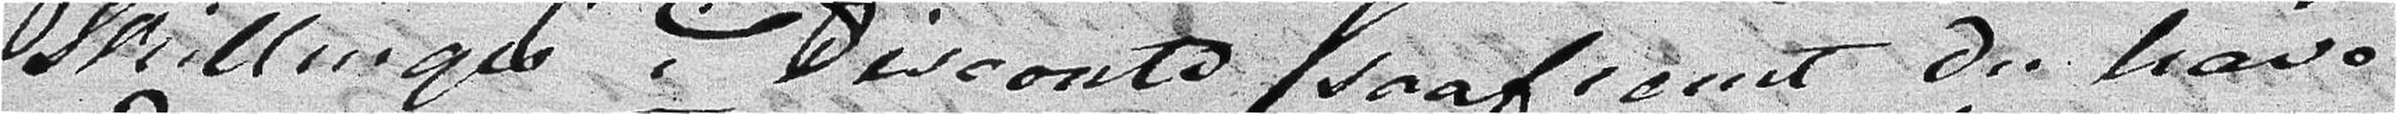Skilling i Disconto saafremt Du have
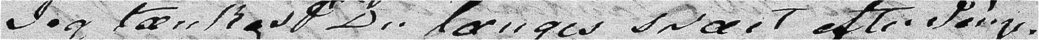Jeg tænker Du længes svært efter Penge,
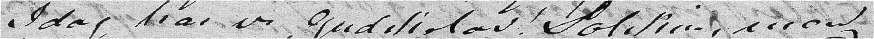Idag har vi Gudskelov Solskin, men
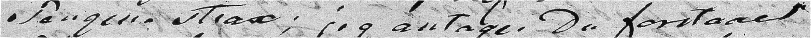Pengene strax; jeg antager Du forstaaer
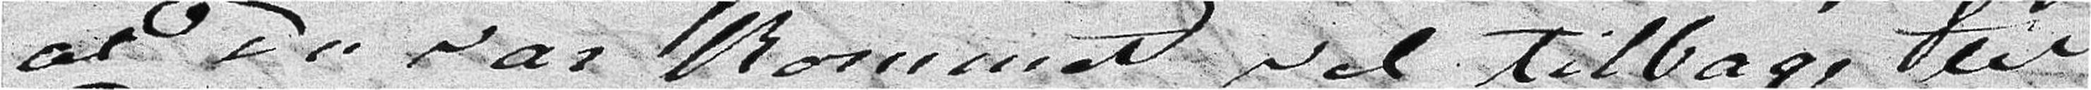at Du var kommet vel tilbage til
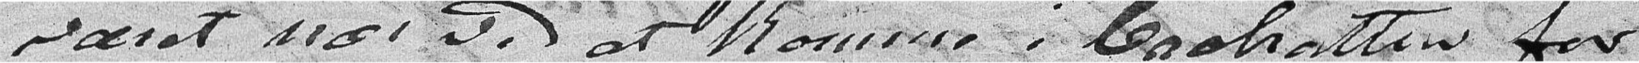været nær ved at komme i Cachotten for
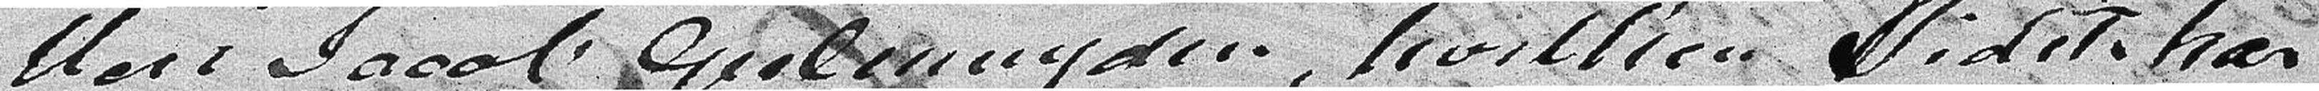Herr Jacob Gielmuyden, hvilken Sidste har
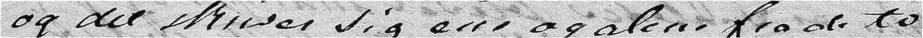og det skriver sig ene og alene fra de to
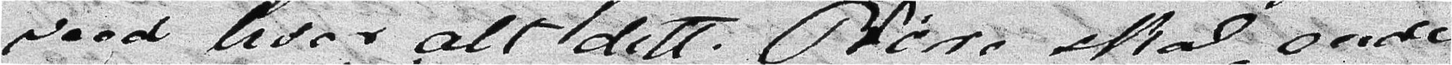veed hvor alt dette Røre skal ende
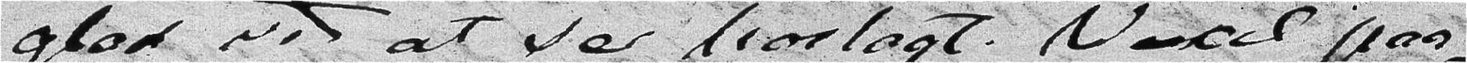glad ved at see hoslagt Vexel paa
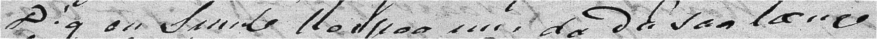Dig en Smule herpaa nu, da Du saa længe
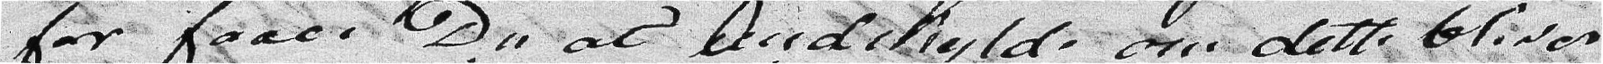for faaer Du at undskylde om dette bliver
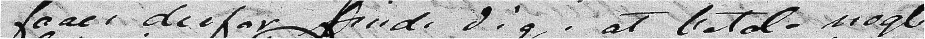faaer derfor finde Dig i at betale nogle
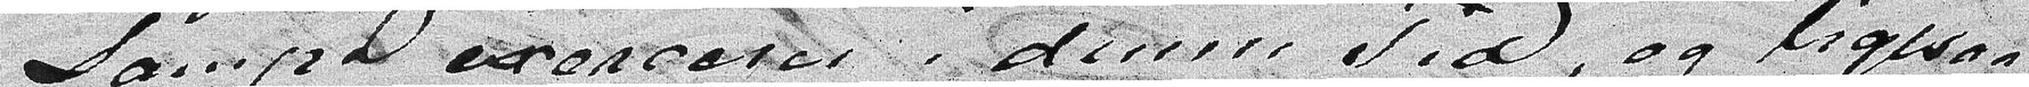Lampe exercerer i denne Tid, og ligesaa
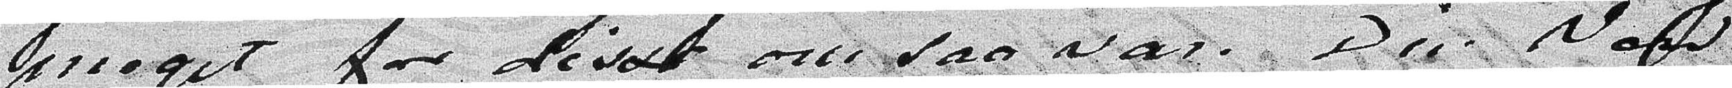meget for disse om saa var. Den Von
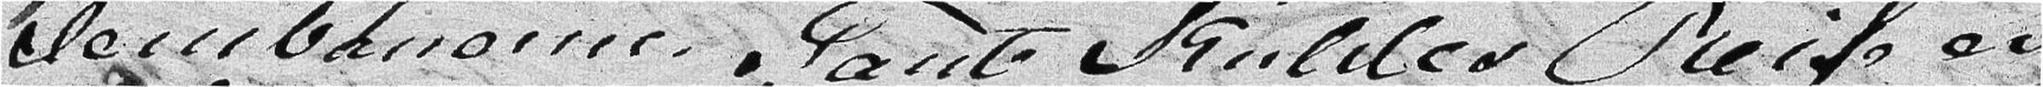cleinbanerne Tante Kühles Reise er
